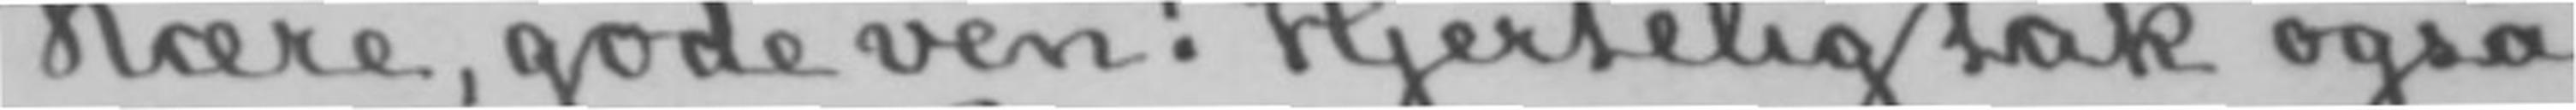Kære, gode ven! Hjertelig tak også
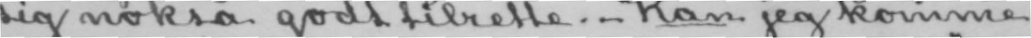sig nokså godt tilrette.- Kan jeg komme
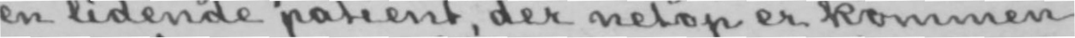en lidende patient, der netop er kommen
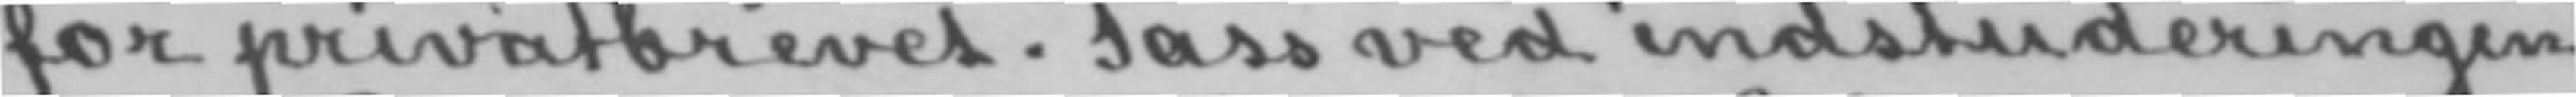for privatbrevet. Pass ved indstuderingen
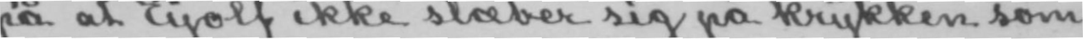på at Eyolf ikke slæber sig på krykken som
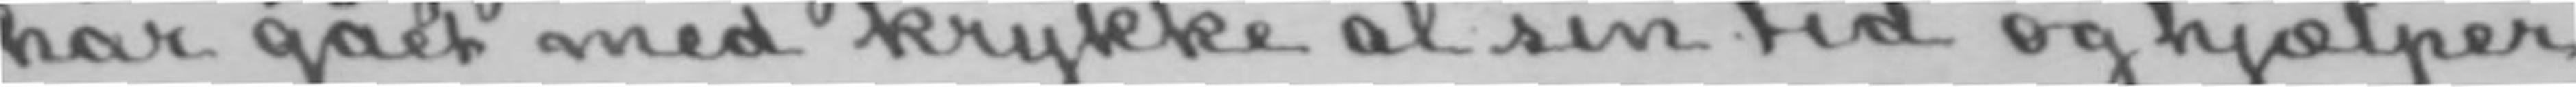har gået med krykke al sin tid og hjælper
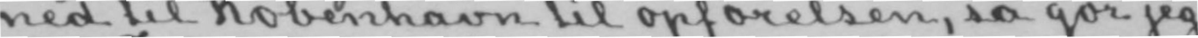ned til København til opførelsen, så gør jeg
